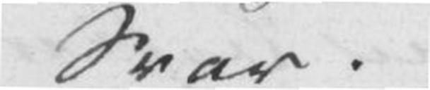Svar.
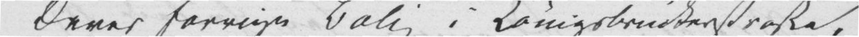Deres forrige Bolig i Königsbrückerstrasse,
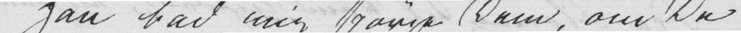Han bad mig spørge Dem, om De
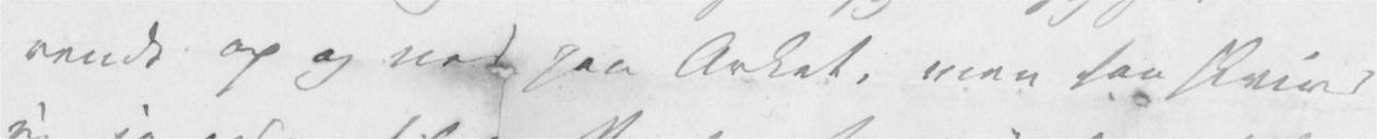vendt op og ned paa Arket, men saa skriver
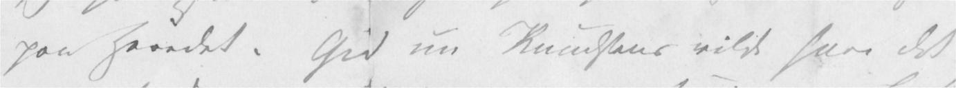paa Hovedet. Gid nu Knudsens vilde have det
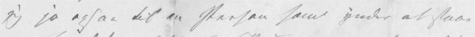jeg jo ogsaa til en Person som ynder at staa
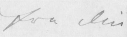fra Din
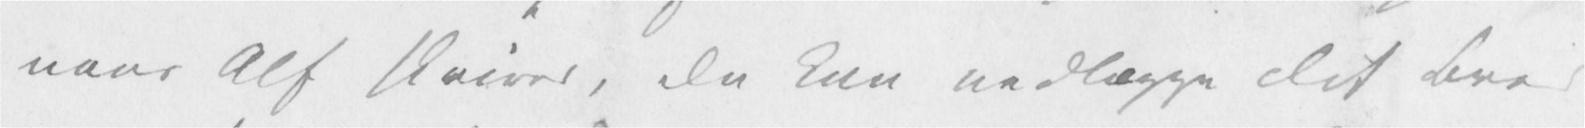naar Alf skriver, Du kan nedlægge Dit Brev
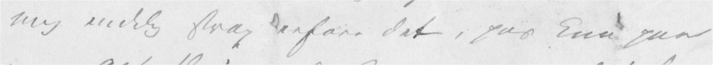mig endelig strax erfare det, pas kun paa
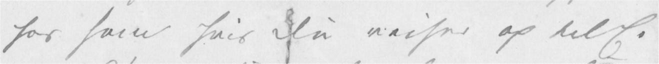hos ham hvis Du reiser op til E.
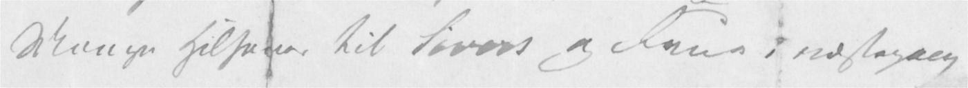Mange Hilsener til Sivers og Frue; næstegang
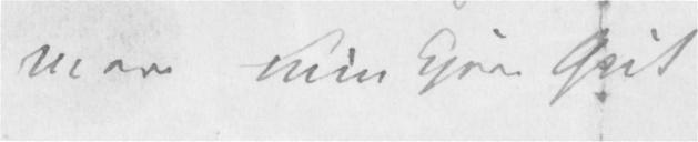mere min kjere Gut
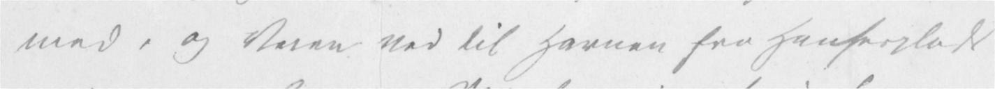med, og Veien ned til Havnen fra Hauserplads
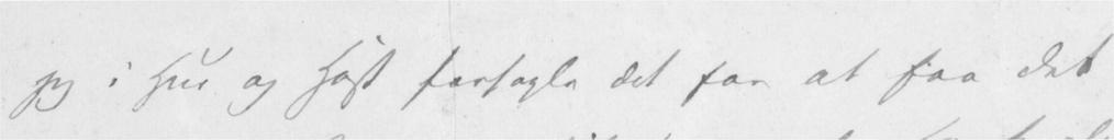jeg i Hui og Hast forsegle det for at faa det
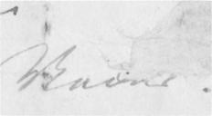Moder.
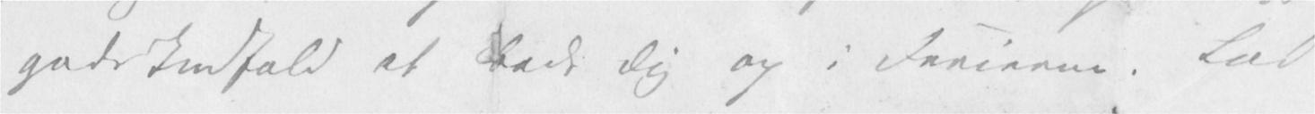gode Indfald at bede Dig op i Ferierne. Lad
